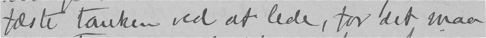fæste tanken ved at lede, for det maa
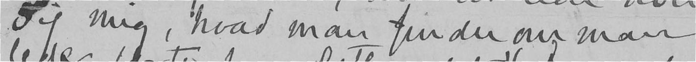Sig mig, hvad man finder om man
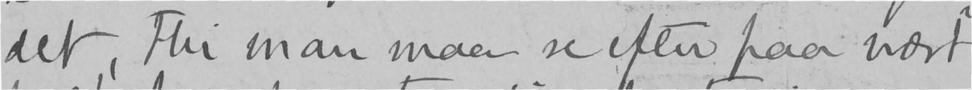det, thi man maa se efter paa nært
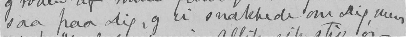saa paa Dig, og vi snakkede om Dig, men
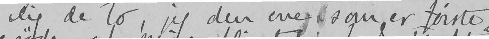Dig de to, jeg den ene, som er første
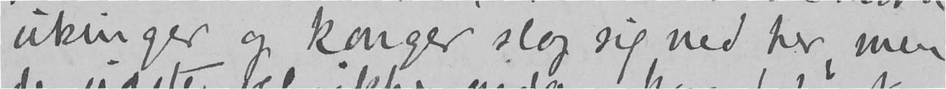vikinger og konger slog sig ned her, men
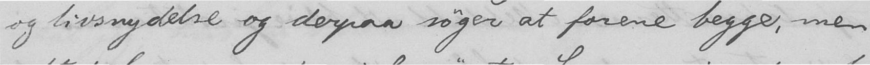og livsnydelse og derpaa søger at forene begge, men
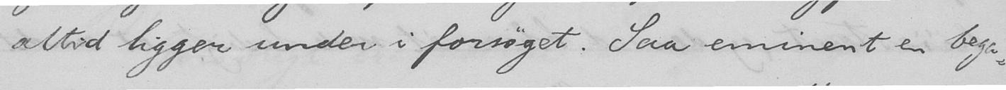altid ligger under i forsøget. Saa eminent en bega-
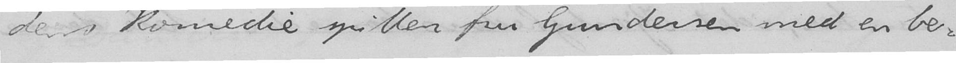dens komedie spiller fru Gundersen med en be-
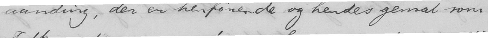aanding, der en henførende og hendes gemal som
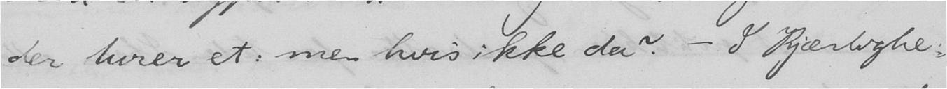der bevrer et: men hvis ikke da? - I Kjærlighe-
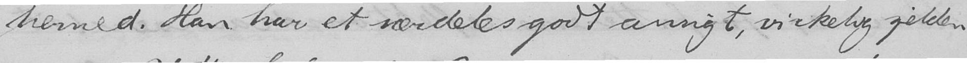herned. Han har et særdeles godt ansigt, virkelig sjelden
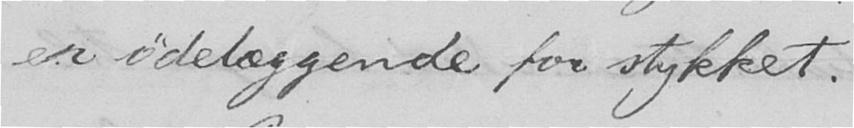er ødelæggende for stykket.
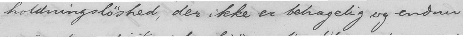holdningsløshed, der ikke er behagelig og endnu
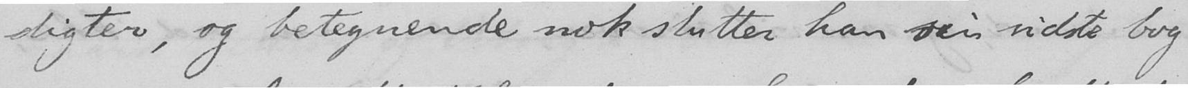digter, og betegnende nok slutter han sin sidste bog
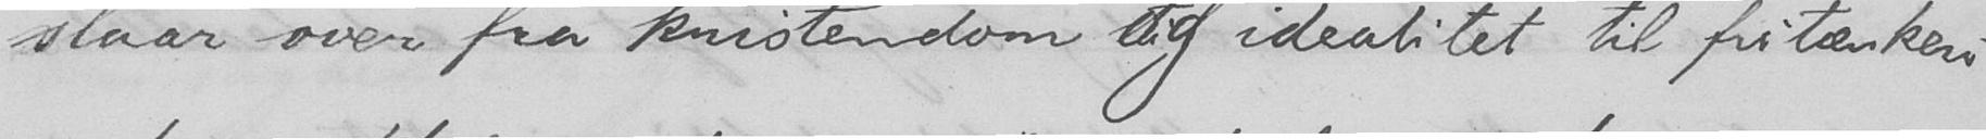slaar over fra kristendom og idealitet til fritænkeri
